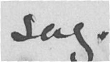sag.
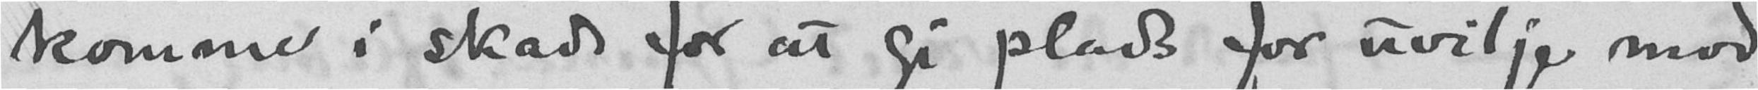komme i skade for at gi plads for uvilje mod
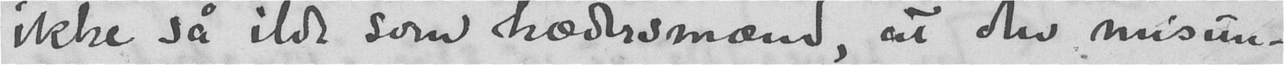ikke så ilde som hædersmænd, at den misun-
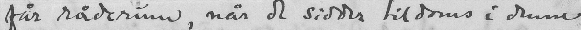får råderum, når de sidder tildoms i denne
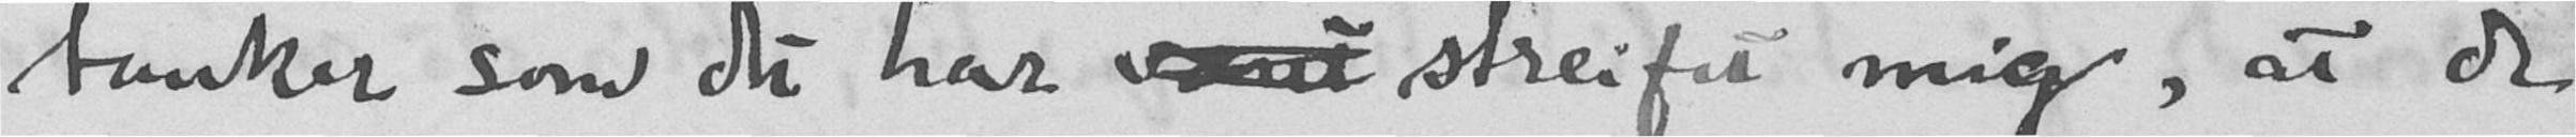tanker som det har været streifet mig, at de
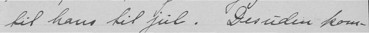til hans til jul. Desuden kom-
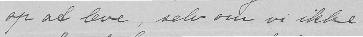op at leve, selv om vi ikke
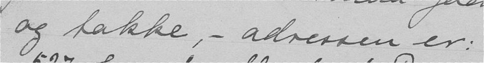og takke, - adressen er:
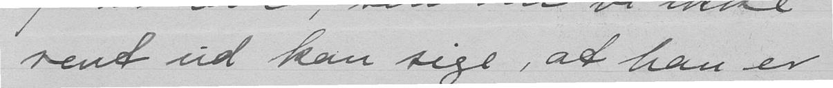rent ud kan sige, at han er
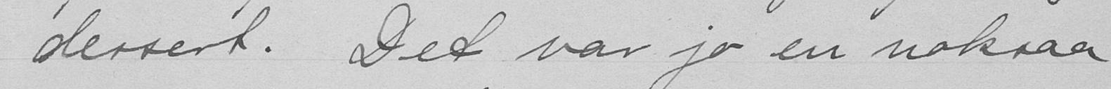dessert. Det var jo en noksaa
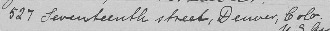527 Seventeenth street, Denver, Colo.
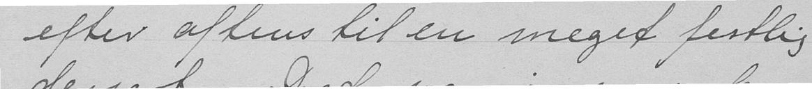efter aftens til en meget festlig
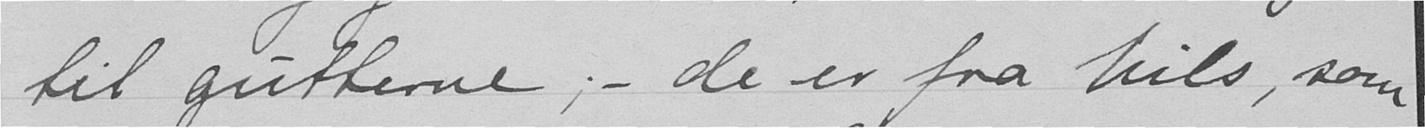til gutterne; - de er fra Nils, som
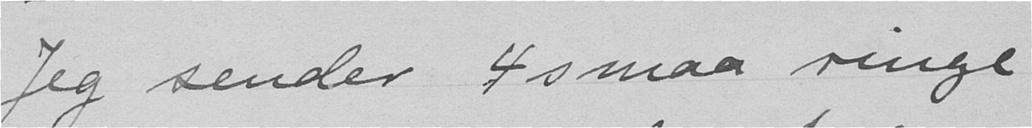Jeg sender 4 smaa ringe
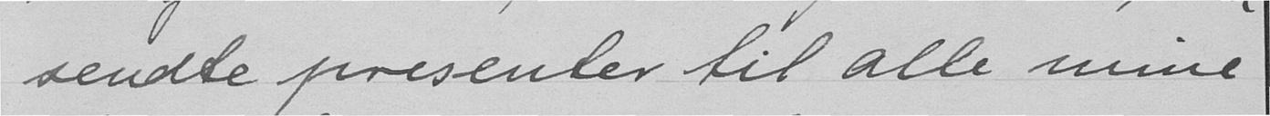sendte presenter til alle mine
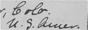U.S. Amer.
